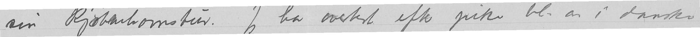sin Kjøbenhavnstur. Jeg har avertert efter piken bl.a. i danske
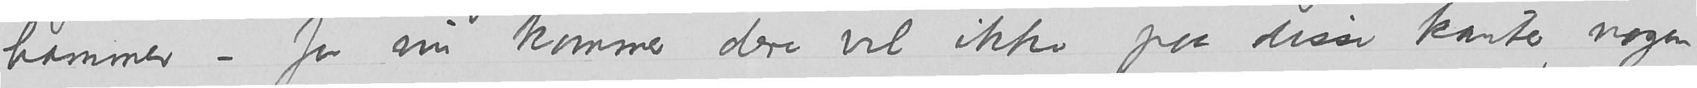hammer – for nu kommer dere vel ikke paa disse kanter nogen
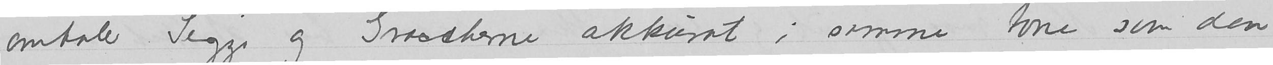omtaler Sigge og Graccherne akkurat i samme tone som den
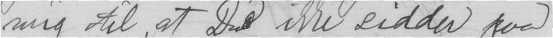mig til, at Du ikke sidder paa
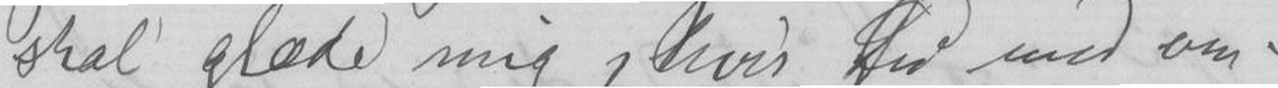skal glæde mig, hvis Du med om-
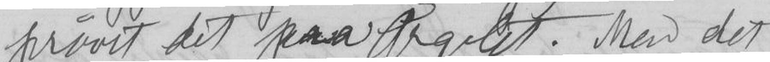prøvet det paa Orgelet. Men det
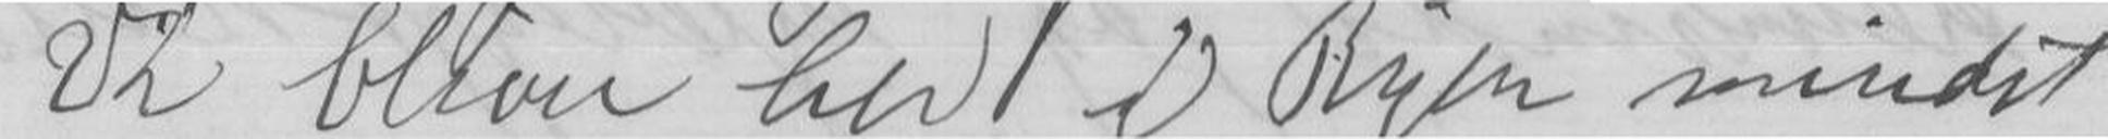Vi bliver her i Byen mindst
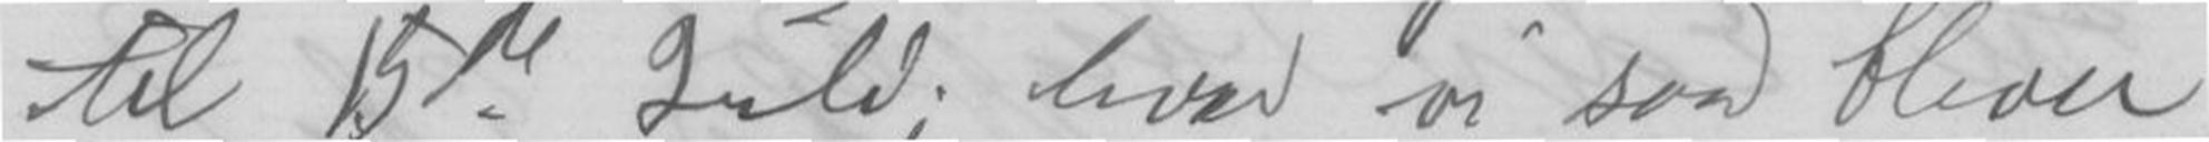til 15de Juli; hvor vi saa bliver
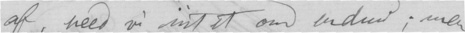af, veed vi intet om endnu; men
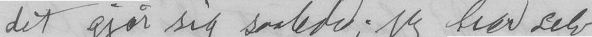det gjør sig saaledes; jeg har selv
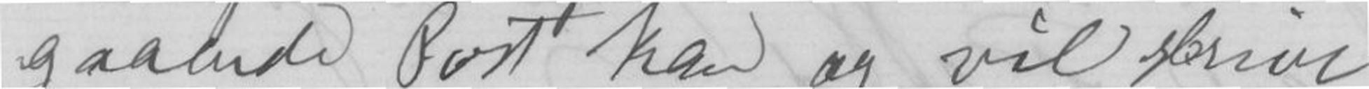gaaende Post kan og vil skrive
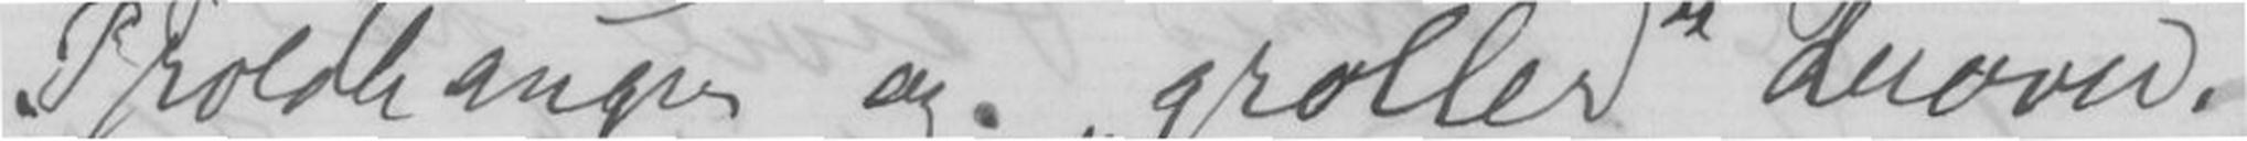Troldhaugen og groller derover.
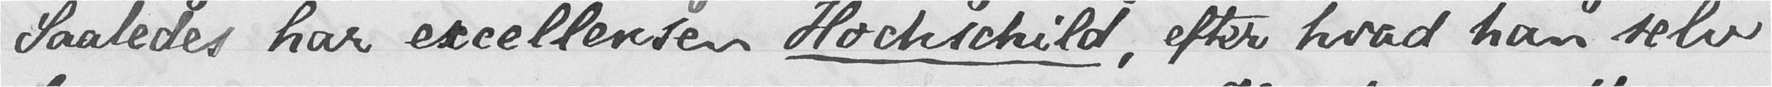Saaledes har excellensen Hochschild, efter hvad han selv
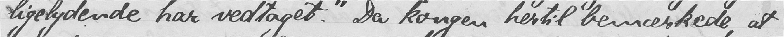ligelydende har vedtaget." Da kongen hertil bemærkede, at
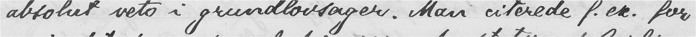absolut veto i grundlovsager. Man citerede f. ex. for
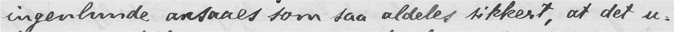ingenlunde ansaaes som saa aldeles sikkert, at det u-
